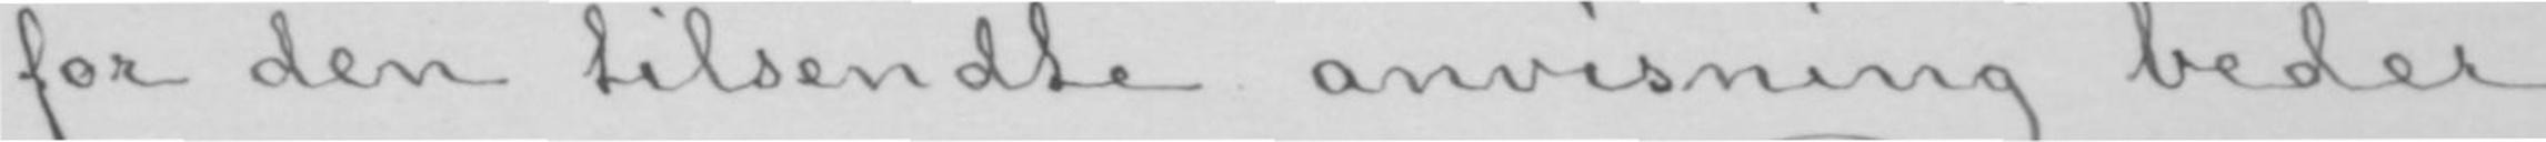for den tilsendte anvisning beder
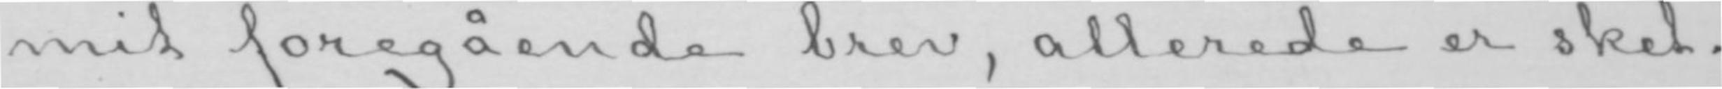mit foregående brev, allerede er sket.
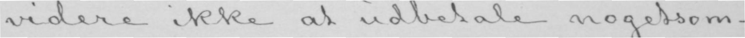videre ikke at udbetale nogetsom-
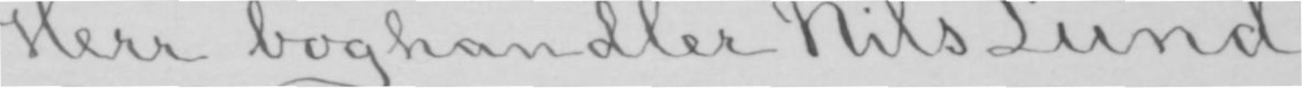Herr boghandler Nils Lund
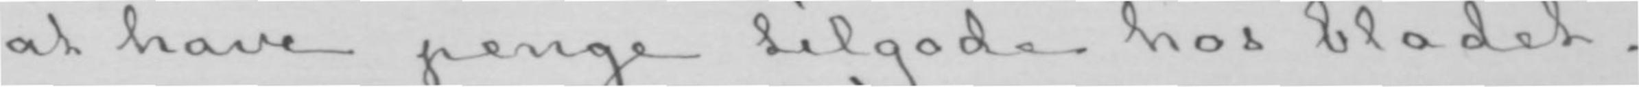at have penge tilgode hos bladet.
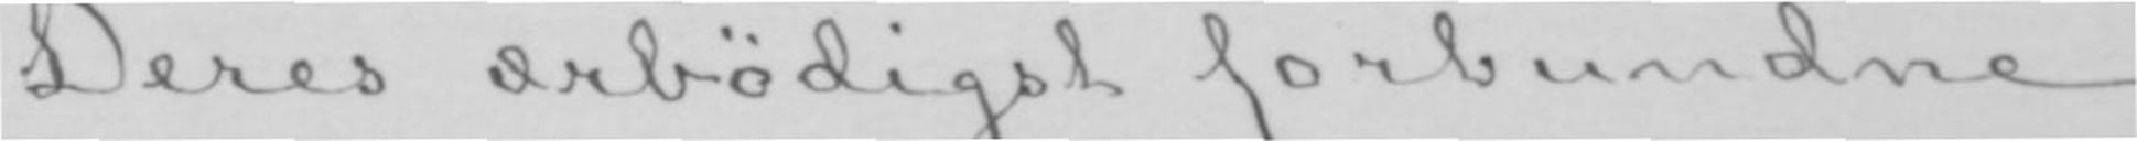Deres ærbødigst forbundne
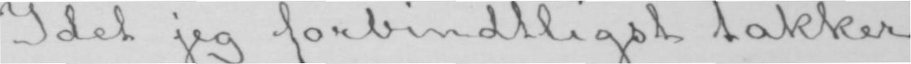Idet jeg forbindtligst takker
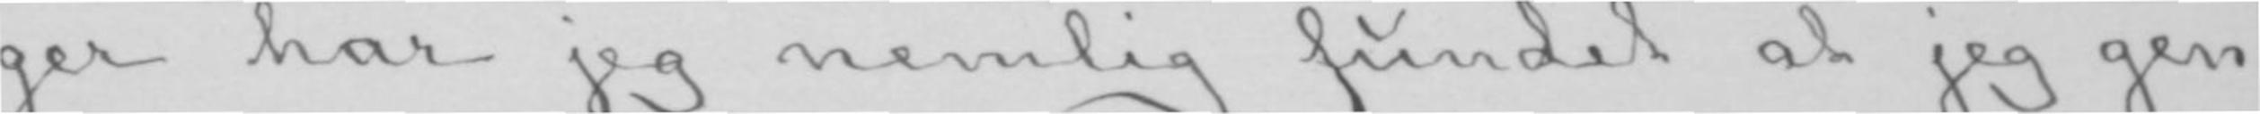ger har jeg nemlig fundet at jeg gen-
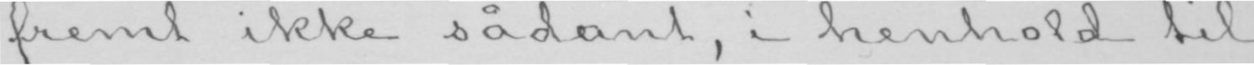fremt ikke sådant, i henhold til
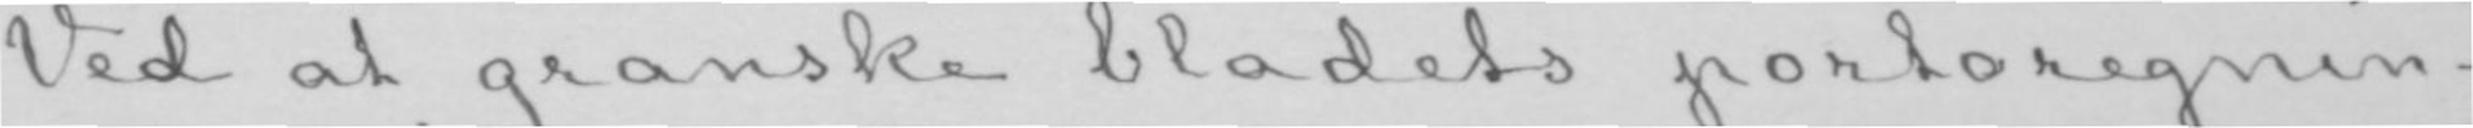Ved at granske bladets portoregnin-
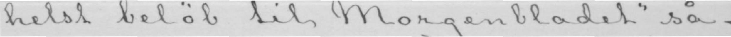helst beløb til «Morgenbladet» så-
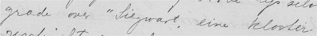græde over "Siegwart, eine kloster-
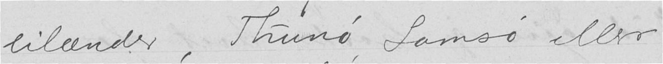eilænder, Thunø, Samsø eller
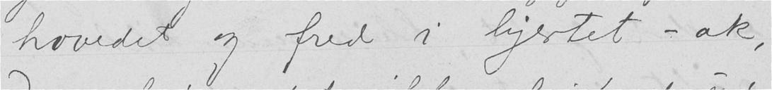hovedet og fred i hjertet - ak,
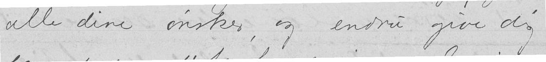alle dine ønsker, og endnu give dig
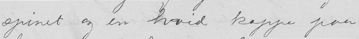spinet og en hvid kappe paa
